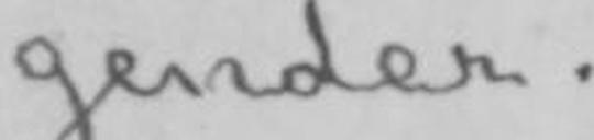gender.
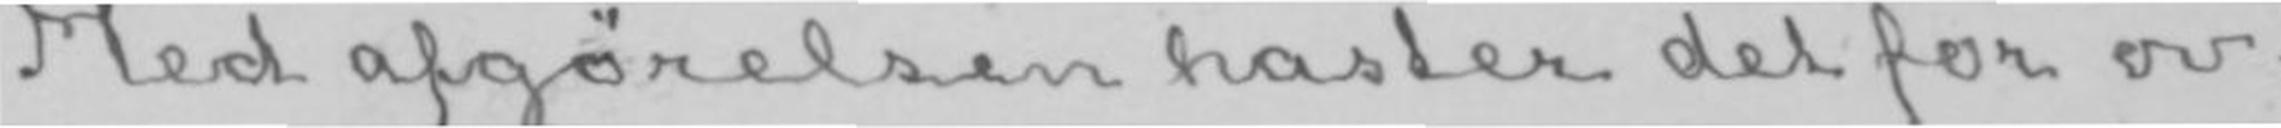Med afgørelsen haster det for øv-
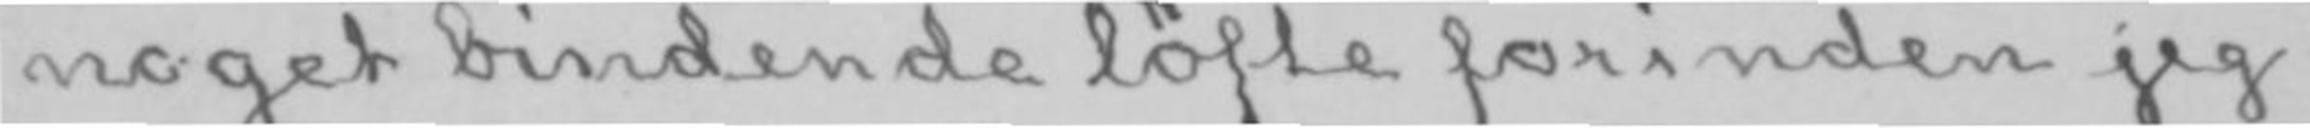noget bindende løfte forinden jeg
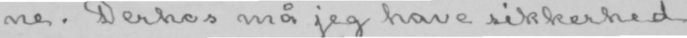ne. Derhos må jeg have sikkerhed
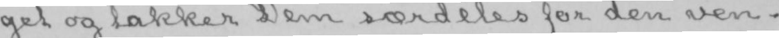get og takker Dem særdeles for den ven-
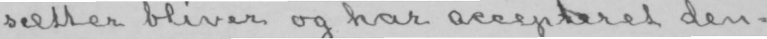sætter bliver og har accepteret den-
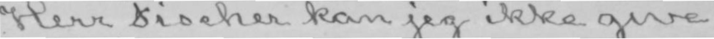Herr Fischer kan jeg ikke give
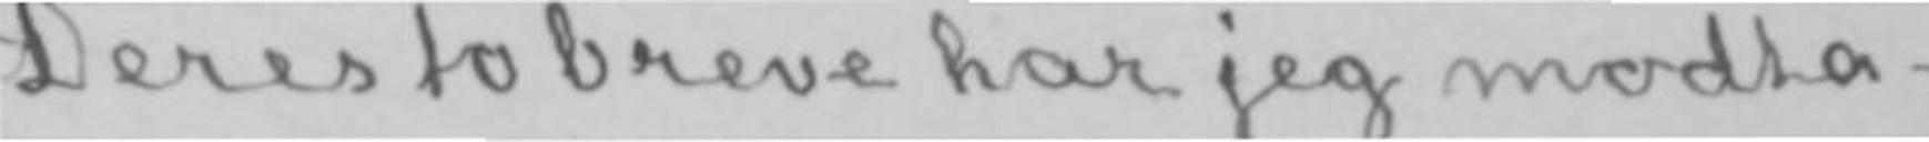Deres to breve har jeg modta-
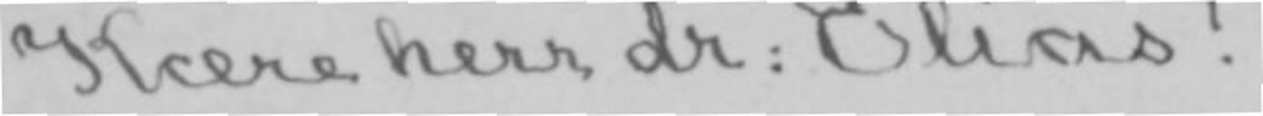Kære herr dr: Elias!
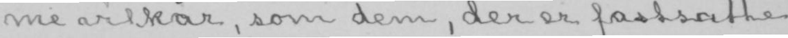me vilkår, som dem, der er fastsatte
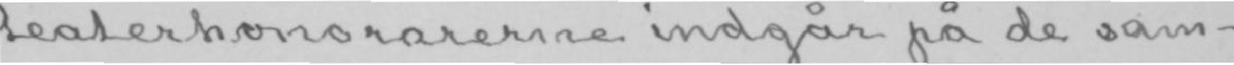teaterhonorarerne indgår på de sam-
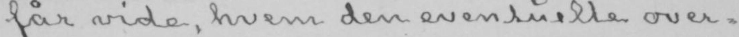får vide, hvem den eventuelle over-
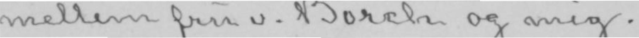mellem fru v. Borch og mig.
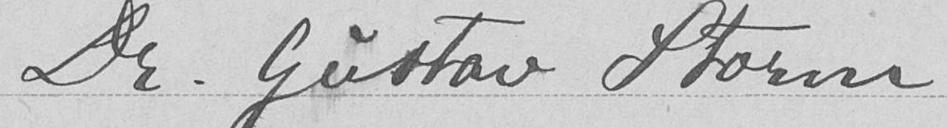Dr. Gustav Storm
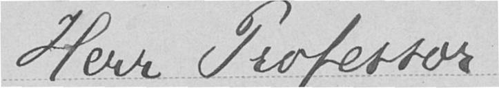Herr Professor
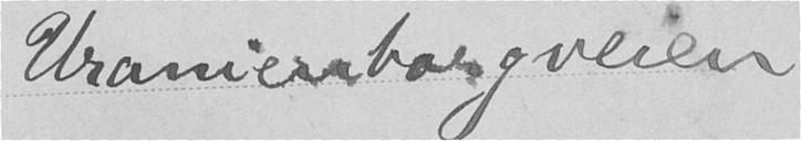Uranienborgveien
